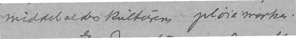middelalderskulturens pløiemarker.
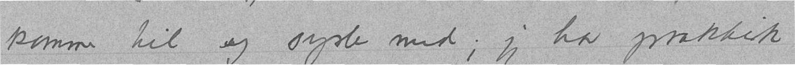komme til og sysle med; jeg har praktisk
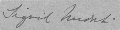Sigrid Undset.
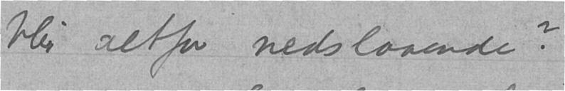blir altfor nedslaaende?
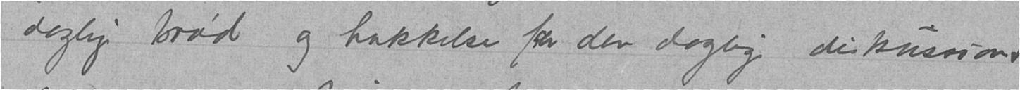daglige brød og hakkelse for den daglige diskussions
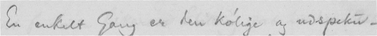En enkelt Gang er den kølige og udspeku-
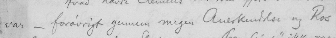var - forøvrigt gennem megen Anerkendelse og Ros
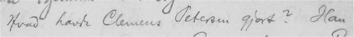Hvad havde Clemens Petersen gjort? Han
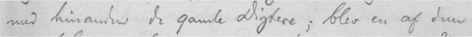med hinanden de gamle Digtere; blev en af dem
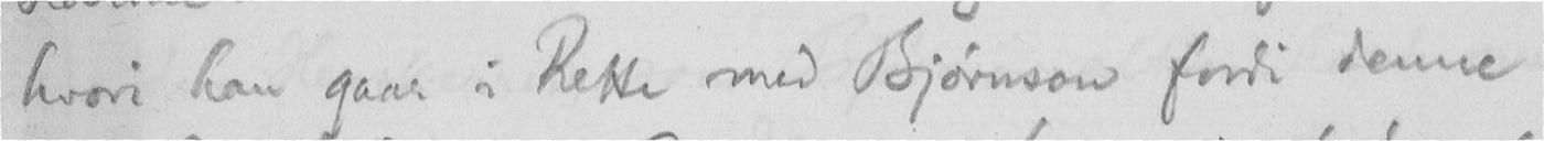hvori han gaar i Rette med Bjørnson fordi denne
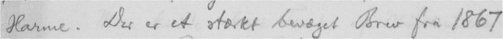Harme. Der er et stærkt bevæget Brev fra 1867
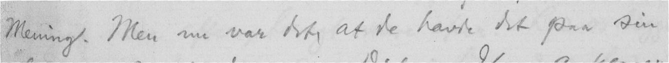Mening. Men nu var det, at de havde det paa sin
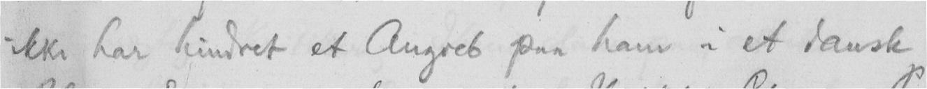ikke har hindret et Angreb paa ham i et dansk
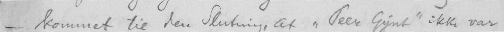- kommet til den Slutning, at "Peer Gynt" ikke var
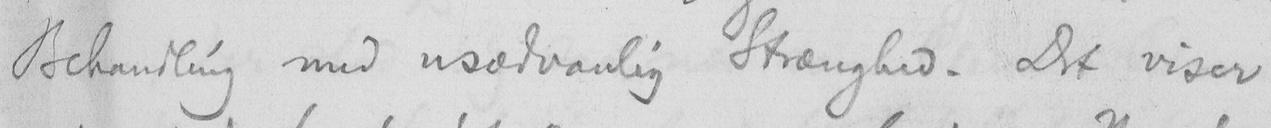Behandling med usædvanlig Strænghed. Det viser
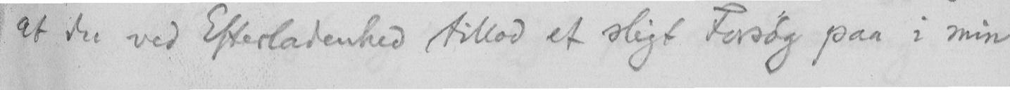at du ved Efterladenhed tillod et sligt Forsøg paa i min
